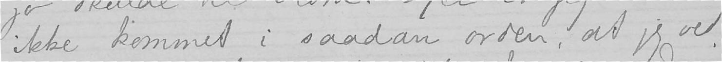ikke kommet i saadan orden, at jeg ved,
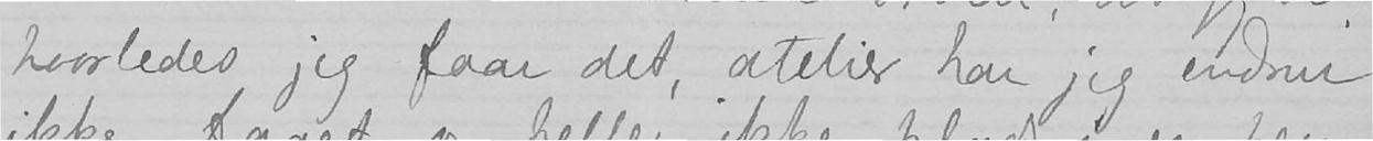hvorledes jeg faar det, atelier har jeg endnu
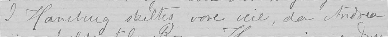I Hamburg skiltes vore veie, da Andrea
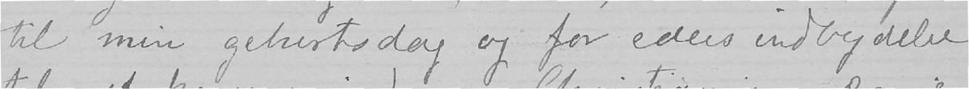til min geburtsdag og tak for eders indbydelse
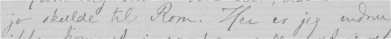jo skulde til Rom. Her er jeg endnu
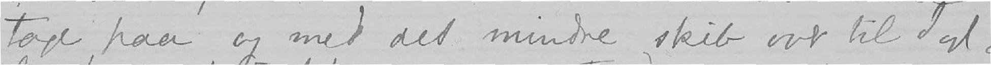tage paa og med det mindre skib over til Jyl-
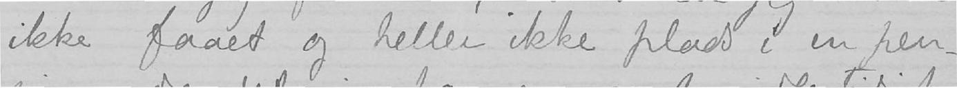ikke faaet og heller ikke plads i en pen-
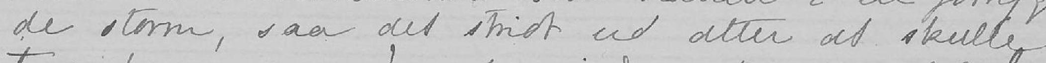de storm, saa det stridt ud atter at skulle
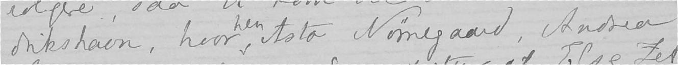drikshavn, hvorhen Asta Nørregaard, Andrea
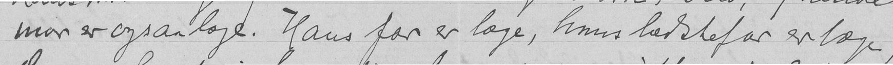mor er ogsaa læge. Hans far er læge, hans bedstefar er læge,
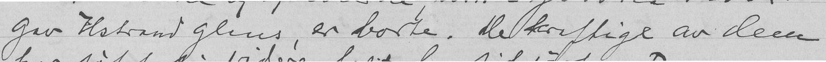gav Hstrand glans, er borte. De kraftige av dem
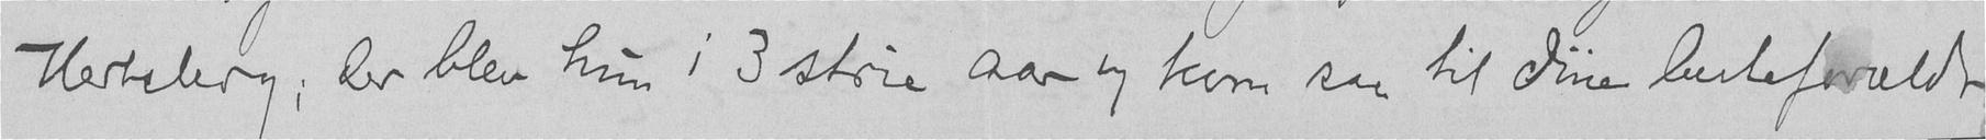Hertzberg; der blev hun i 3 strie aar og kom saa til dine besteforældres
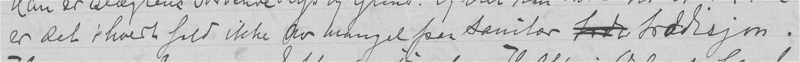er det i hvert fald ikke av mangel paa sanitær  tradisjon.
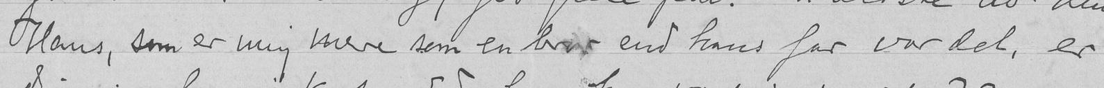Hans, som er mig mere som en bror end hans far var det, er
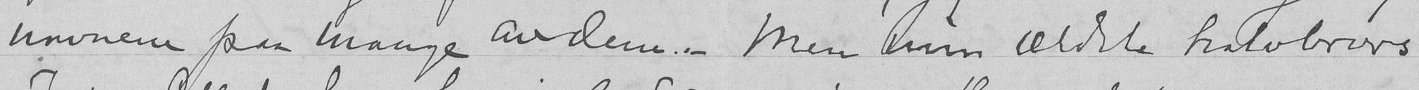navnene paa mange av dem. - Men min ældste halvbrors
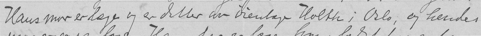Hans mor er læge og er datter av øienlæge Holth i Oslo, og hendes
